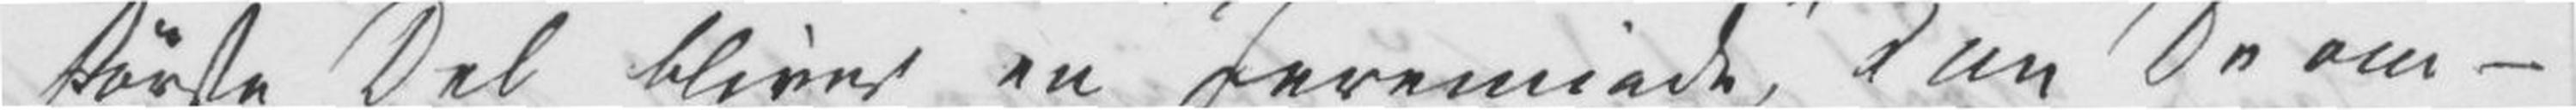største Del bliver en «Jeremiade», kan De om¬
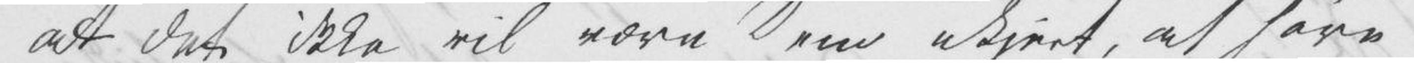at det ikke vil være Dem ukjert, at høre
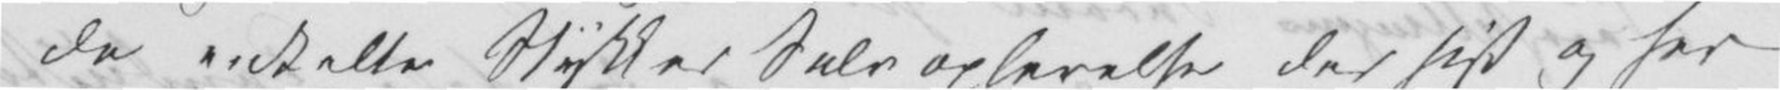de enkelte Stykker Selvoplevelse der hist og her
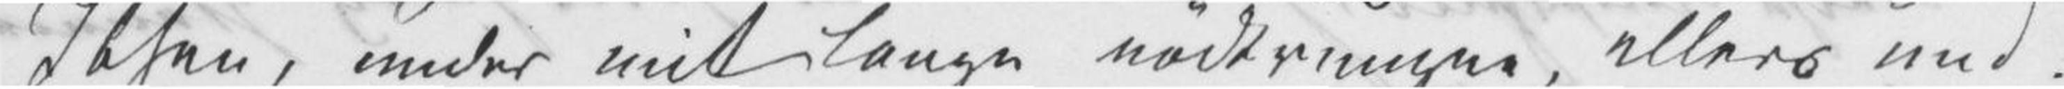Ibsen, under mit lange nødtvungne, ellers uud¬
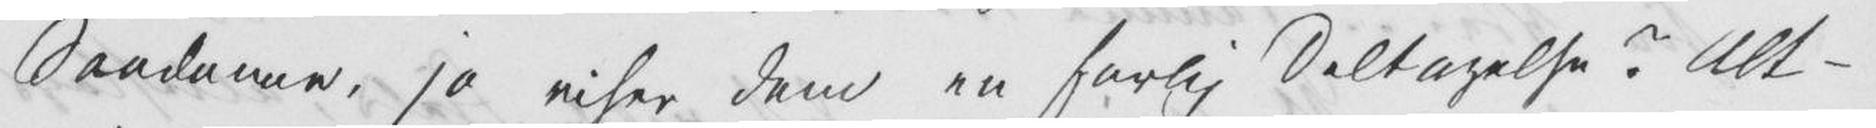Saadanne, ja viser dem en farlig Deltagelse? Alt¬
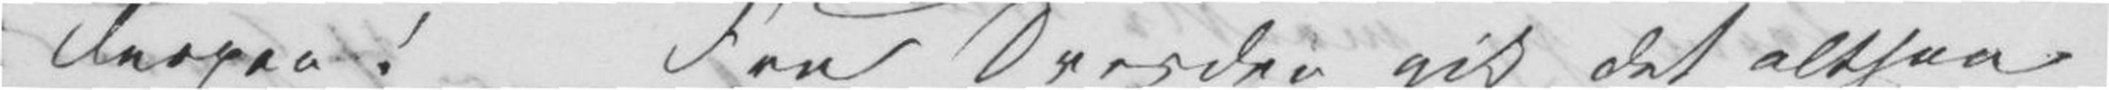derpaa! Fra Dresden gik det altsaa
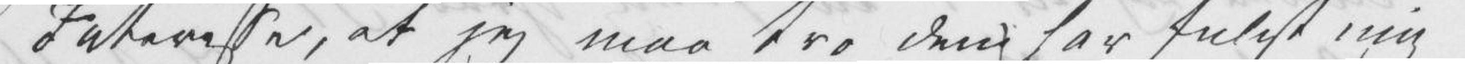Interesse, at jeg maa tro den har fulgt mig
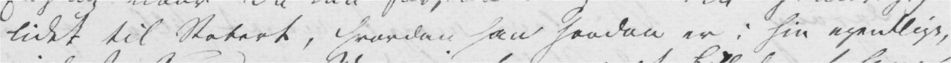lidet til Robert, hvordan han saadan er i sin egentlige,
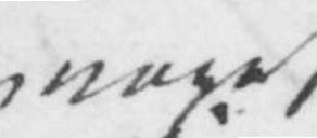noget
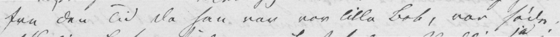fra den Tid da han var vor lille Bob, vor søde,
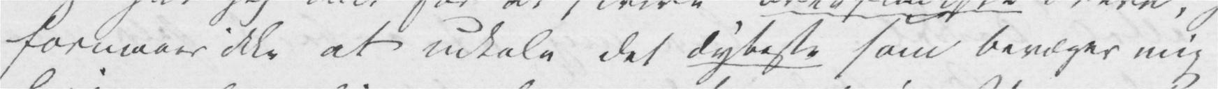formaaer ikke at udtale det dybeste som bevæger mig
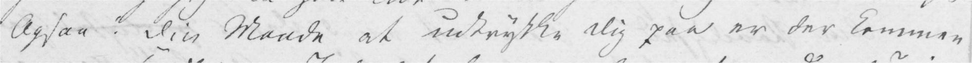Ogsaa i Din Maade at udtrykke Dig paa er der kommen
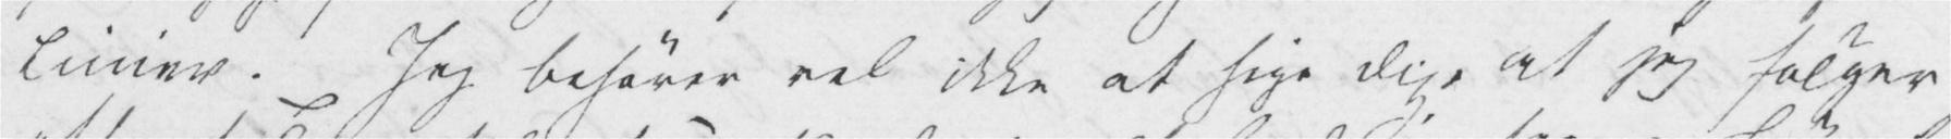Linier. Jeg behøver vel ikke at sige Dig, at jeg følger
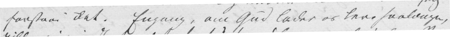forstaae det. Engang, om Gud lader os leve saalænge,
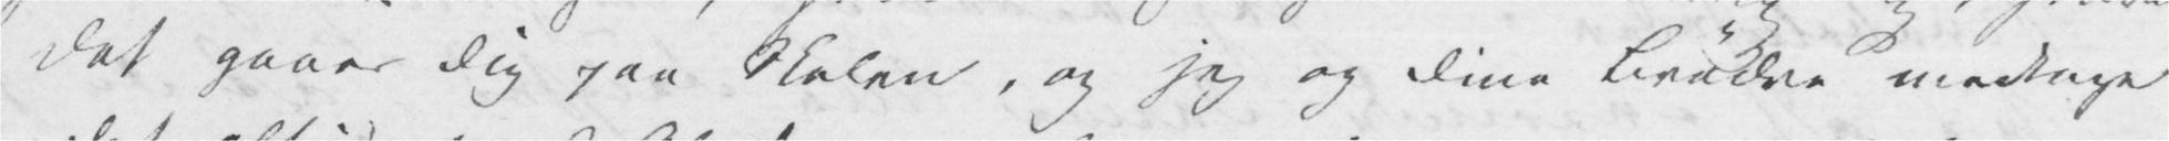det gaaer Dig paa Skolen, og jeg og Dine Brødre modtage
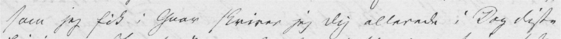som jeg fik i Gaar skriver jeg Dig allerede i Dag disse
